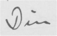Din
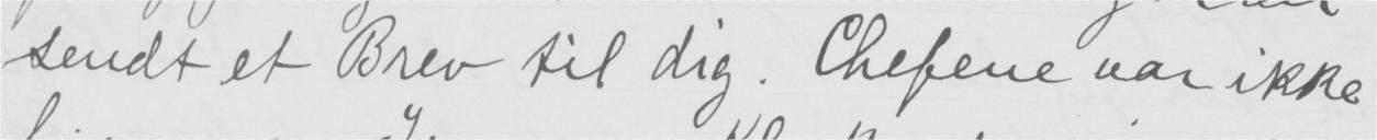sendt et Brev til dig. Chefene var ikke
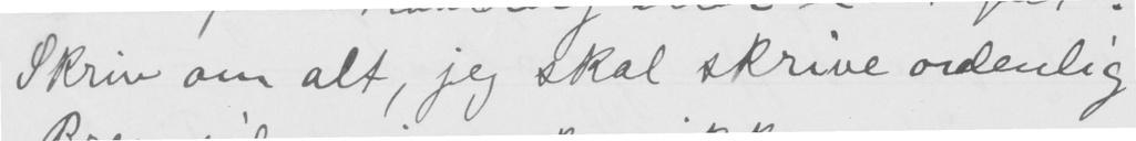Skriv om alt, jeg skal skrive ordenlig
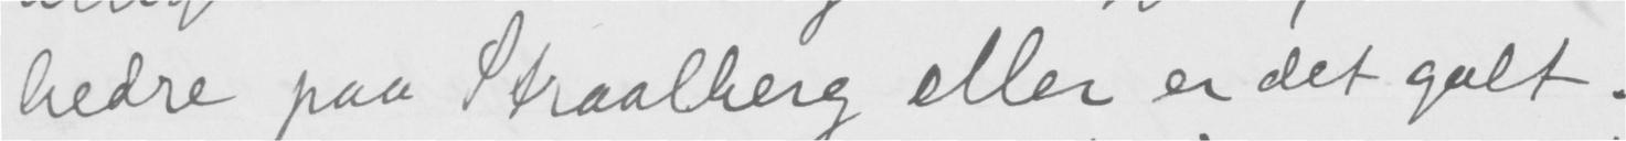bedre paa Straalberg eller er det galt.
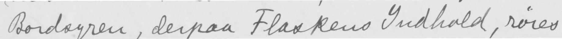Bordsgren, derpaa Flaskens Indhold, røres
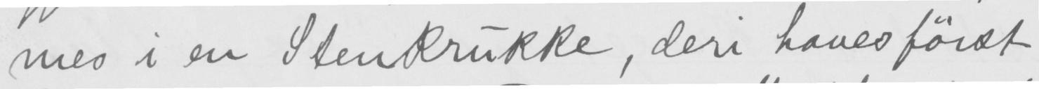mes i en Stenkrukke, deri haves først
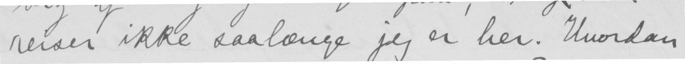reiser ikke saalænge jeg er her. Hvordan
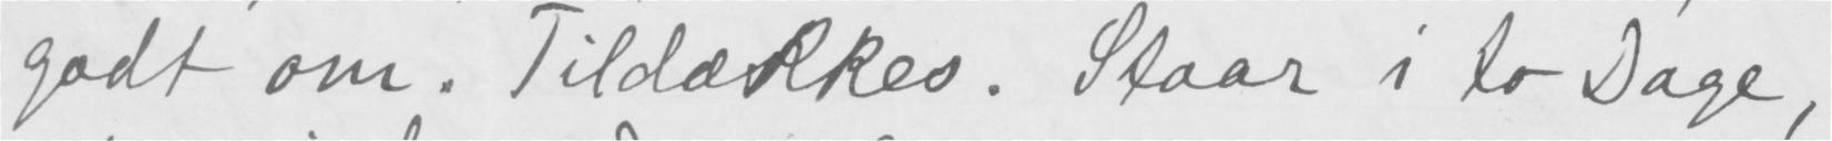godt om. Tildækkes. Staar i to Dage,
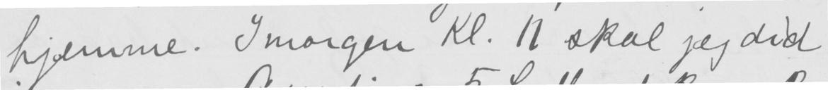hjemme. Imorgen Kl. 11 skal jeg did
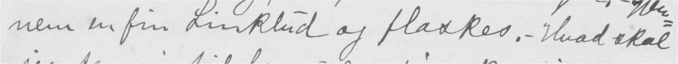nem en fin Linklud og flaskes. — Hvad skal
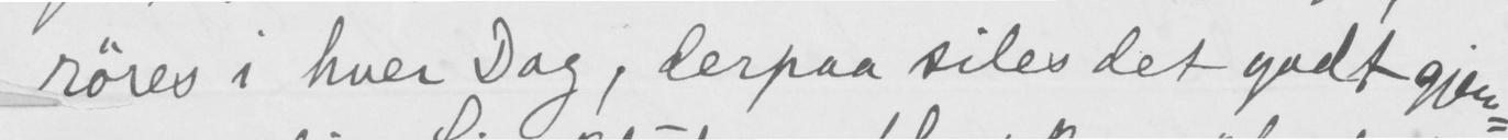røres i hver Dag, derpaa siles det godt gjen-
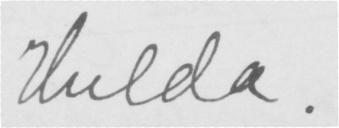Hulda.
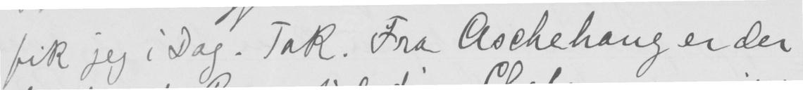fik jeg i Dag. Tak. Fra Aschehoug er der
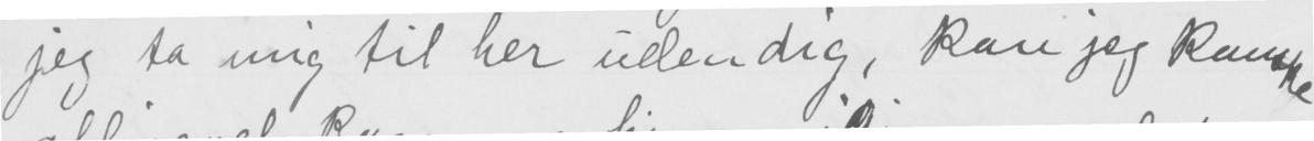jeg ta mig til her uden dig, kan jeg kanske
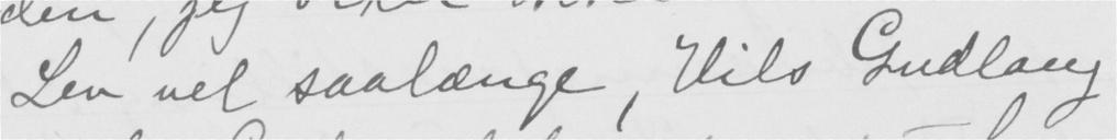Len vel saalænge, hils Gudlaug
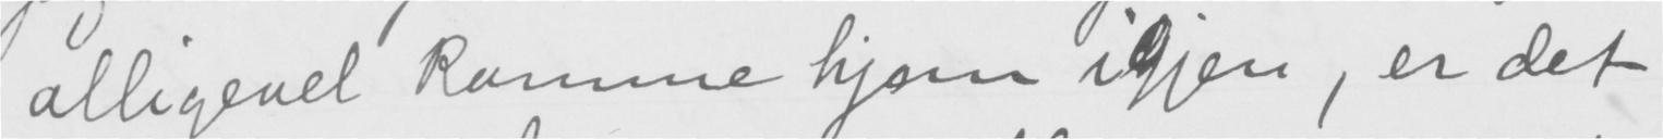alligevel komme hjem igjen, er det
